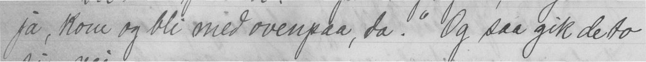ja, kom og bli med ovenpaa, da." Og saa gik de to
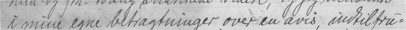i mine egne betragtninger over en avis, indtil fru-
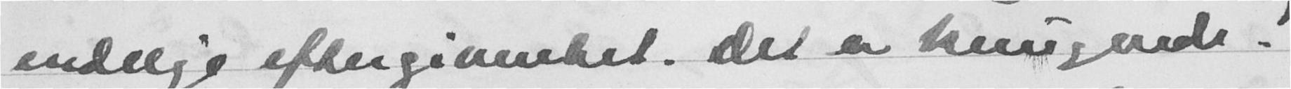endelige eftergivenhet. Det er knugende!
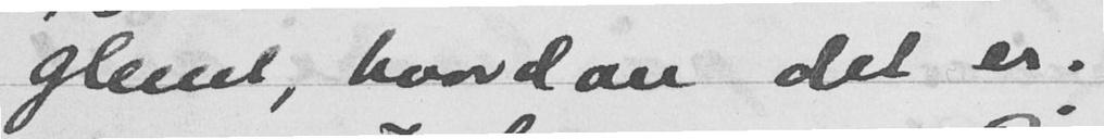glemt, hvordan det er.
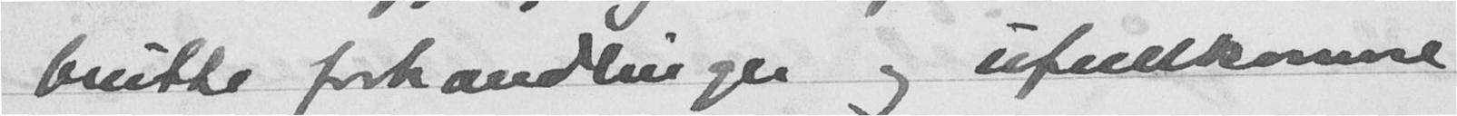og brutte forhandlinger og ufullkomne
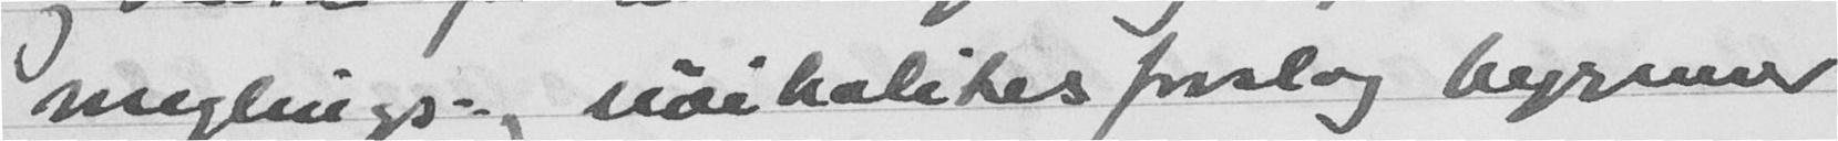meglings- og nøitralitetsforslag begynner
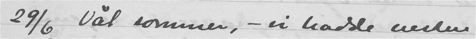29/6 Våt sommer, - vi hadde nesten
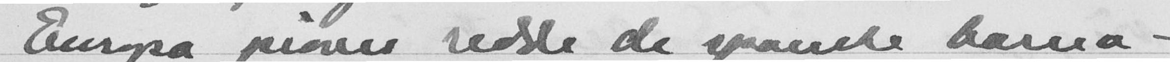Europa prøver redde de spanske barna -
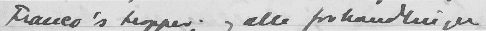Franco's tropper; og alle forhandlinger
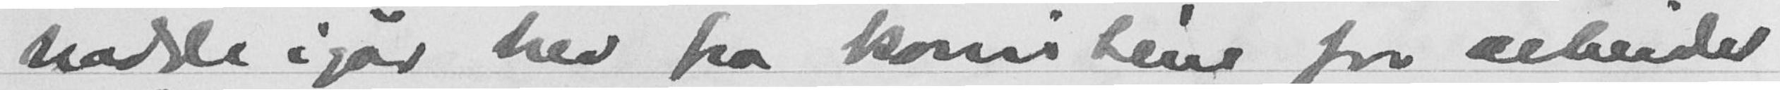hadde igår brev fra komitéen for arbeide
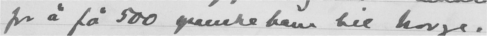for å få 500 spanske barn til Norge.
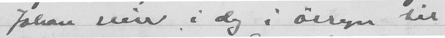Johan reiser i dag i øsregn til
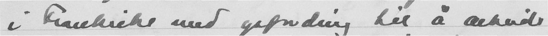i Frankrike med opfording til å arbeide
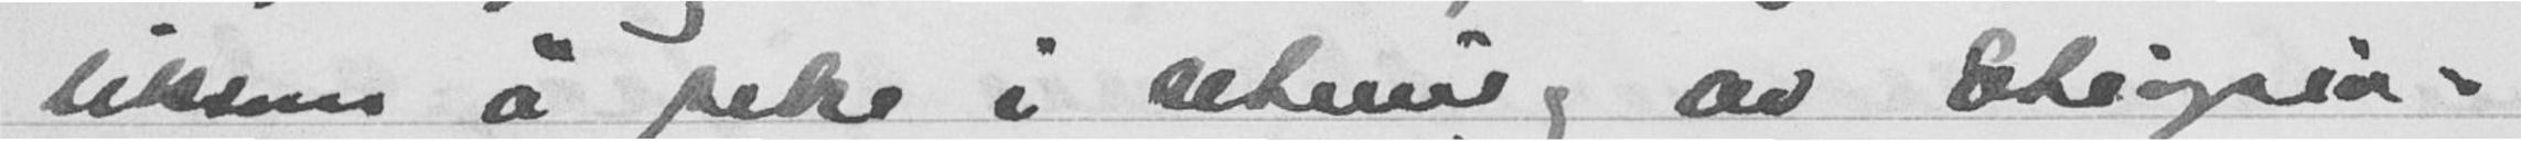liksom å peke i retning av Etiopia,
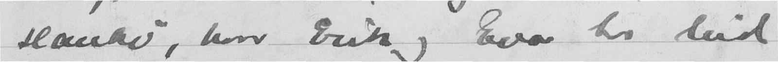Hankø, hvor Erik og Eva hos hiid
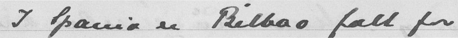I Spania er Bilbao falt for
